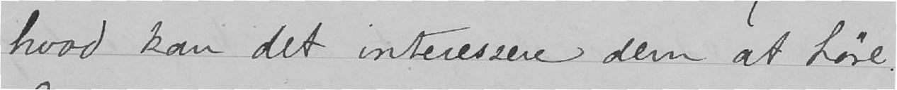hvad kan det interessere dem at høre.
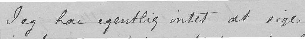Jeg har egentlig intet at sige
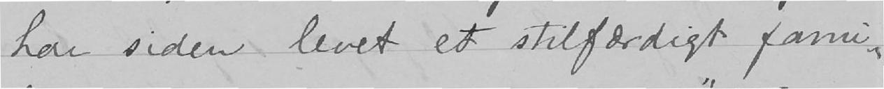har siden levet et stilfærdigt fami-
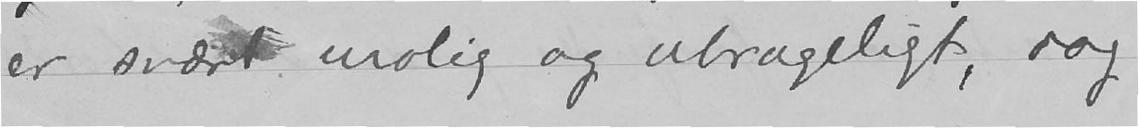er svært urolig og ubrugeligt, dog
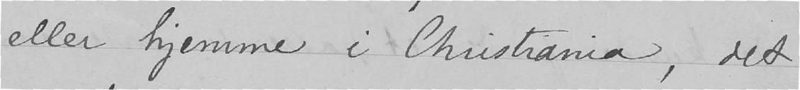eller hjemme i Christiania, det
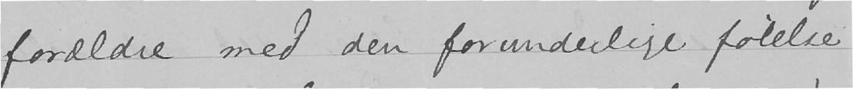forældre med den forunderlige følelse
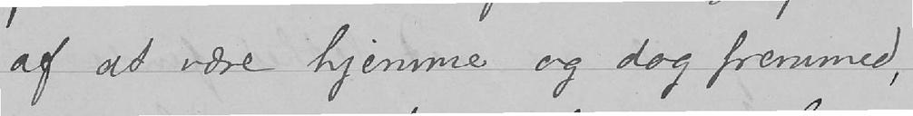af at være hjemme og dog fremmed,
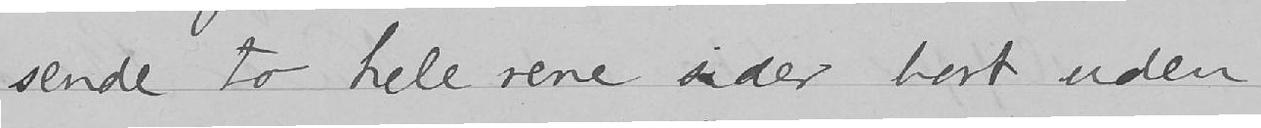sende to hele rene sider bort uden
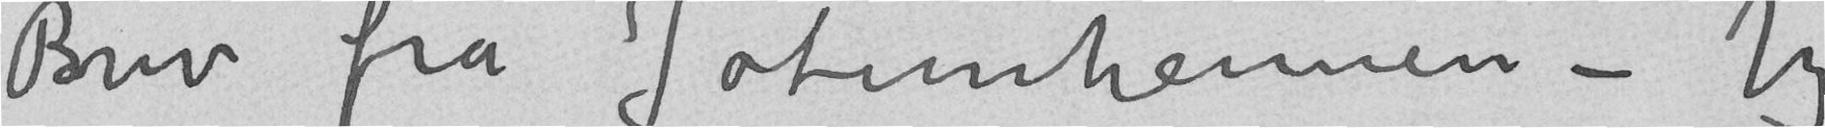Brev fra Jotunheimen – Jeg
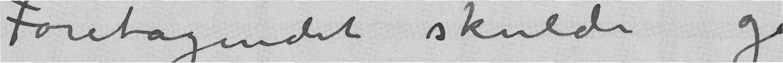Foretagendet skulde gå
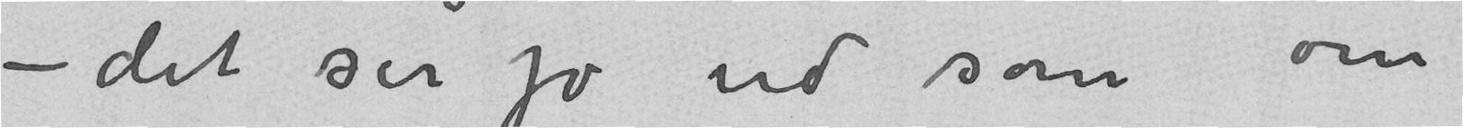– det ser jo ud som om
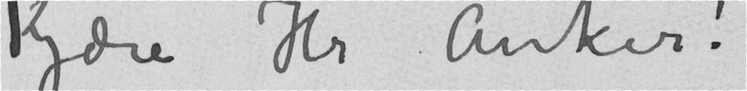Kjære Hr Anker!
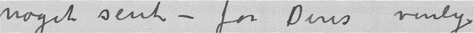noget sent – for Deres venlige
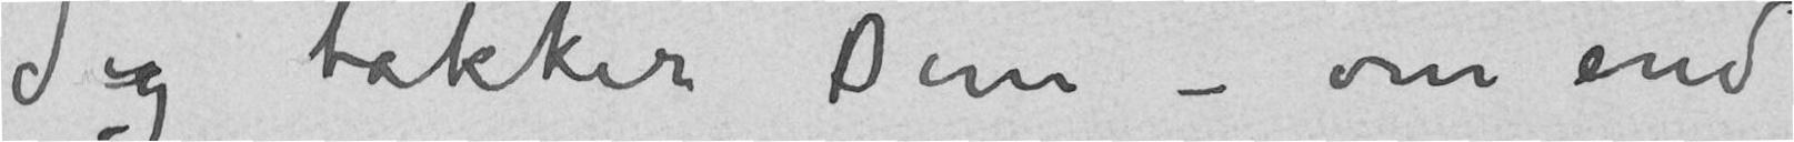Jeg takker Dem – om end
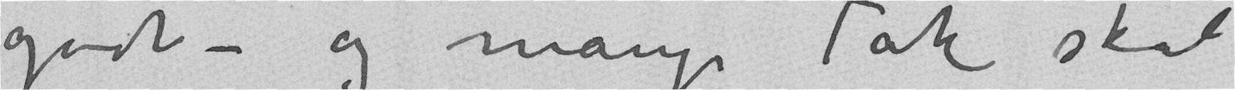godt – og mange Tak skal
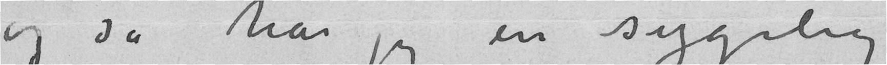og så har jeg en sygelig
